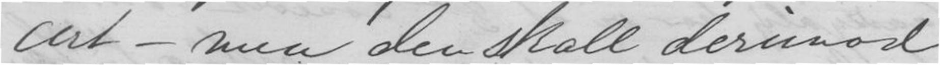cert - men den skall derimod
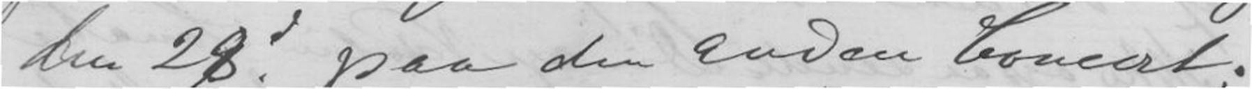den 28d paa den anden Cocert;
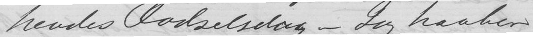hendes Fødselsdag - Jeg haaber
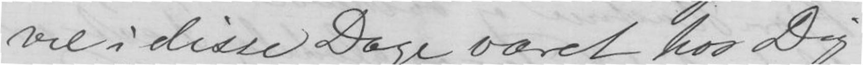vel i disse Dage været hos Dig
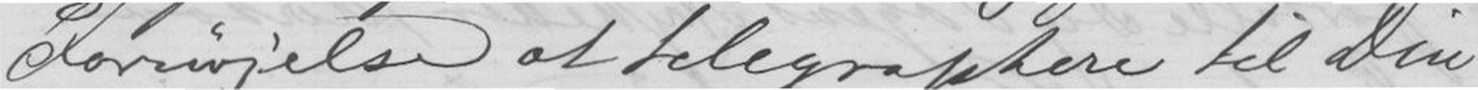Fornøjelse at telegraphere til Din
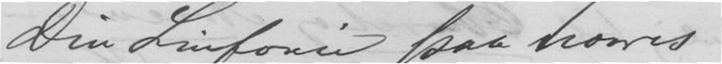Din Sinfonie paa hvores
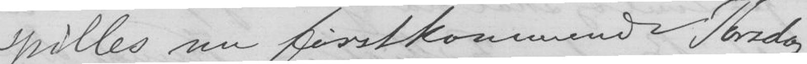spilles nu førstkommende Thorsdag
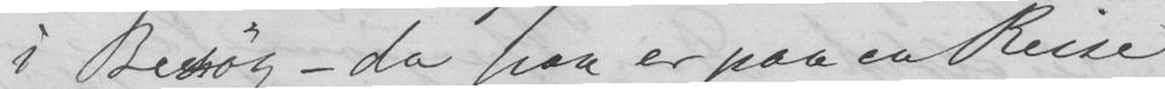i Besøg - da han paa en Reise
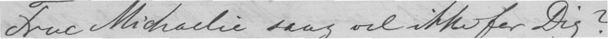Frue Michaelie sang vel ikke for Dig?
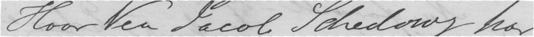Hvor Ven Jakob Schediwy har
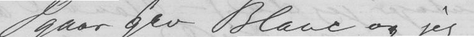Igaar gav Blanc og jeg
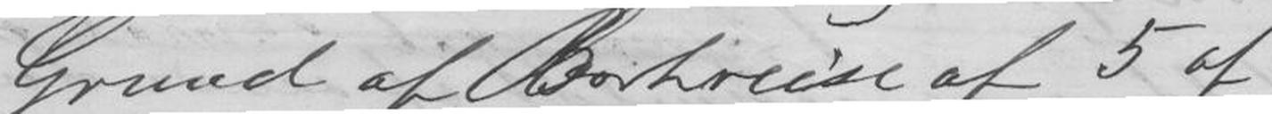Grund af Bortreise af 5 af
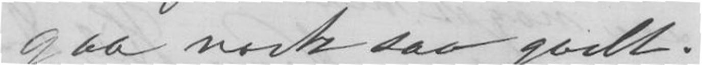gaa nock saa godt.
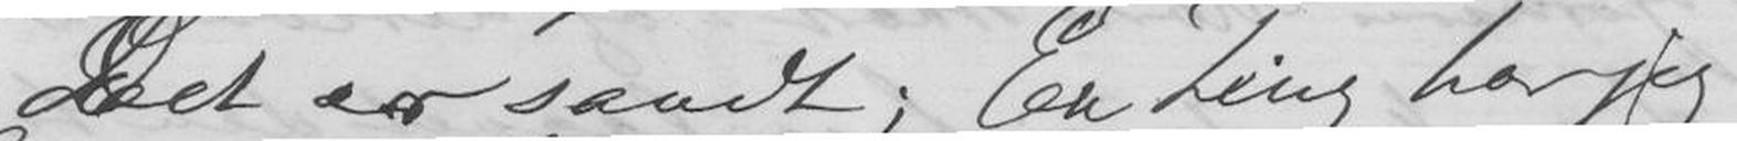Det er sandt; En Ting har jeg
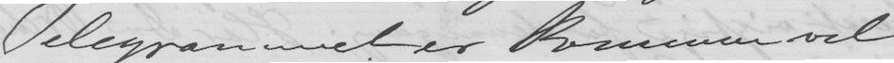Telegrammet er kommen vel
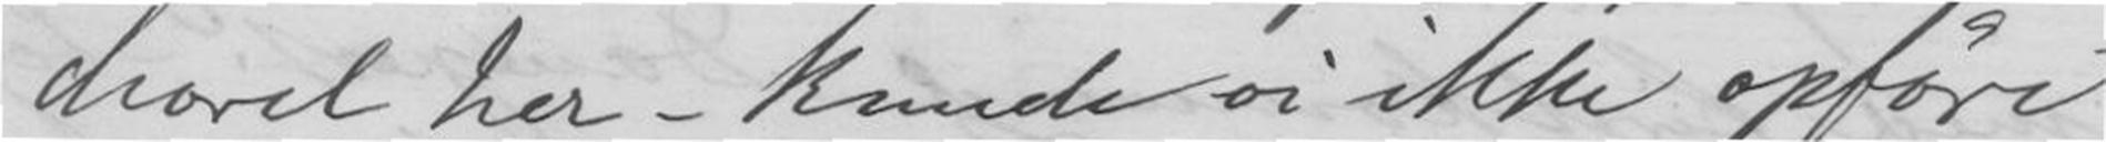choret her - kunde vi ikke opføre
